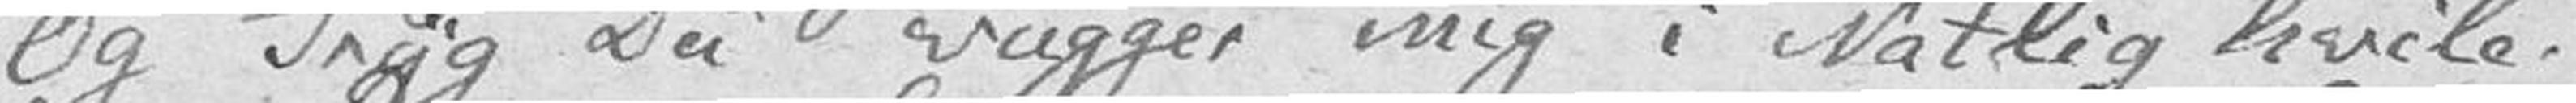Og Tryg Du vugger mig i Natlig hvile.
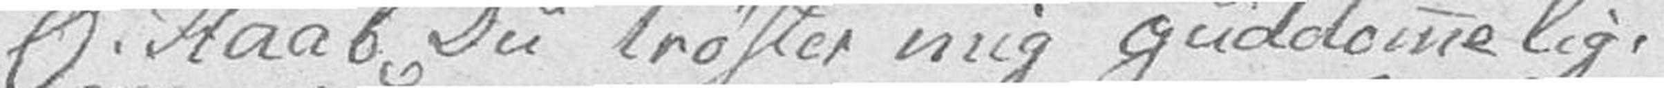O! Haab Du trøster mig guddommelig.
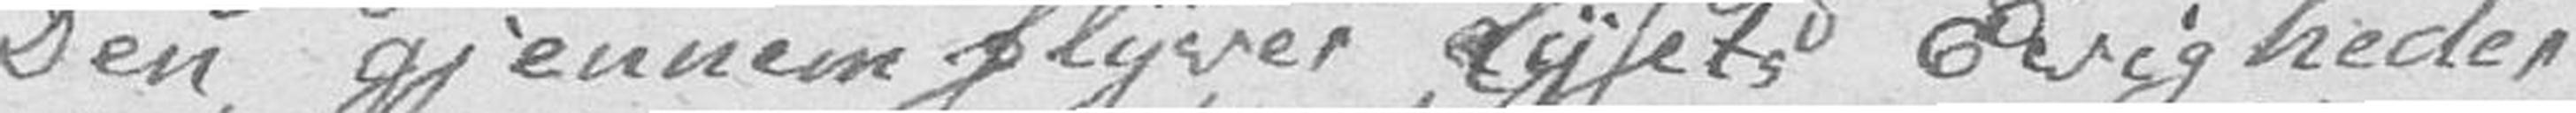Den gjennemflyver Lysets Evigheder
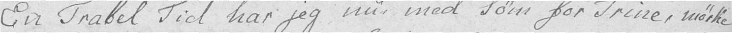En Trabel Tid har jeg nu med Søm for Trine, mørke
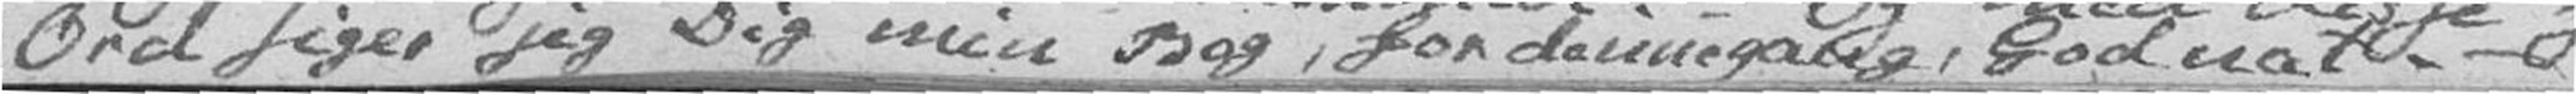Ord sige jeg Dig min Bog, for dennegang, God nat. –
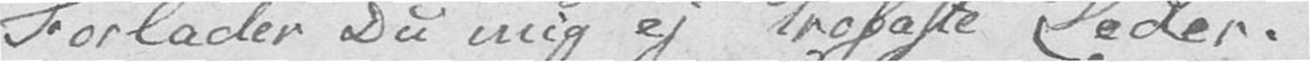Forlader Du mig ej trofaste Leder.
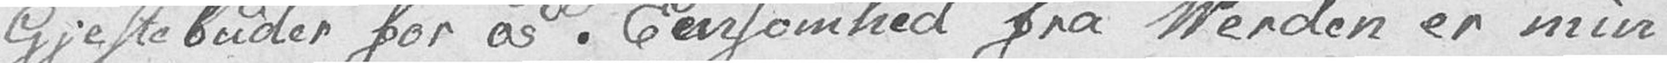Gjestebuder for os. Eensomhed fra Verden er min
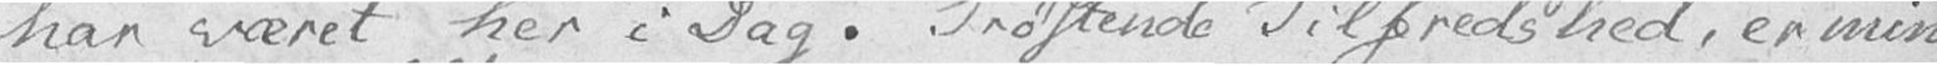har været her i Dag. Trøstende Tilfredshed, er min
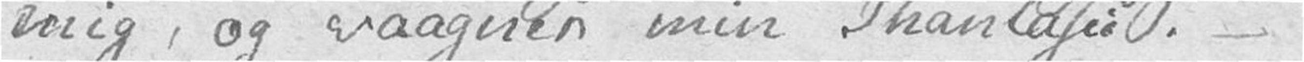mig, og vaagner min Phantasie. –
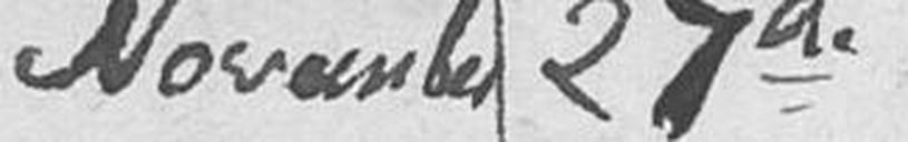November 27de
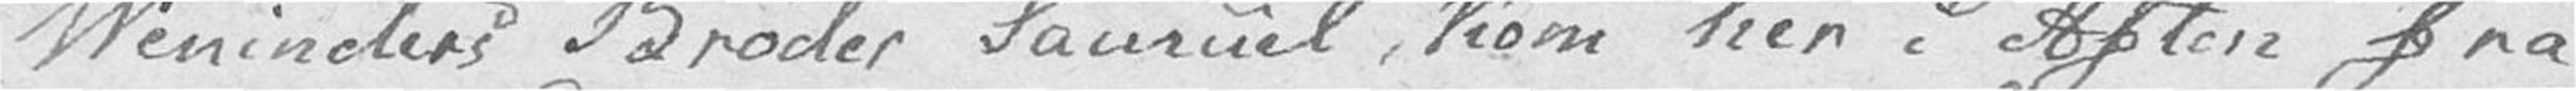Veninders Broder Samuel, kom her i Aften fra
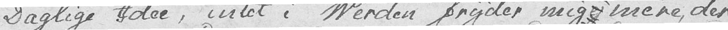Daglige Idee, intet i Verden fryder mig  mere, der
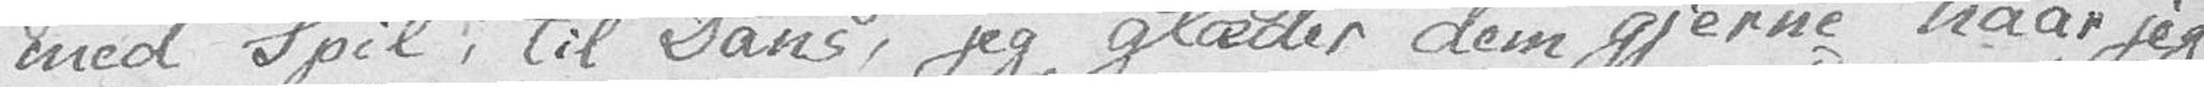med Spil, til Dans, jeg glæder dem gjerne naar jeg
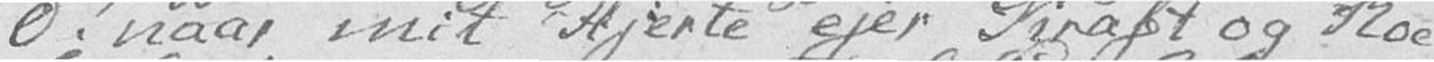O! naar mit Hjerte ejer Kraft og Roe
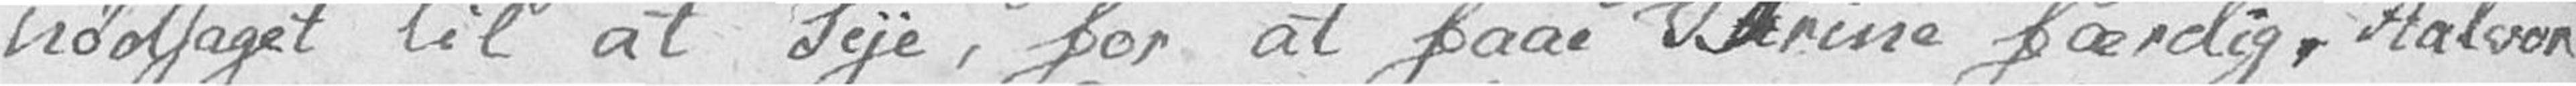nødsaget til at Sye, for at faae Thrine færdig. Halvor
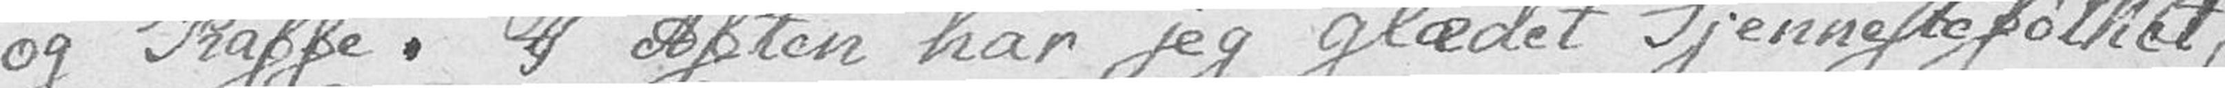og Kaffe. I Aften har jeg glædet Tjennestefolket,
